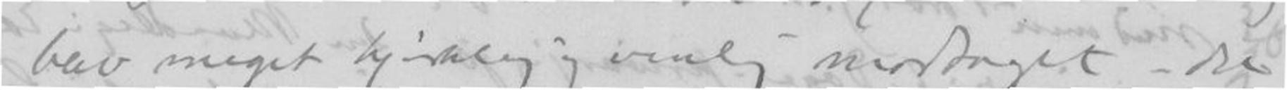og blev meget hjertelig og venlig modtaget. Det
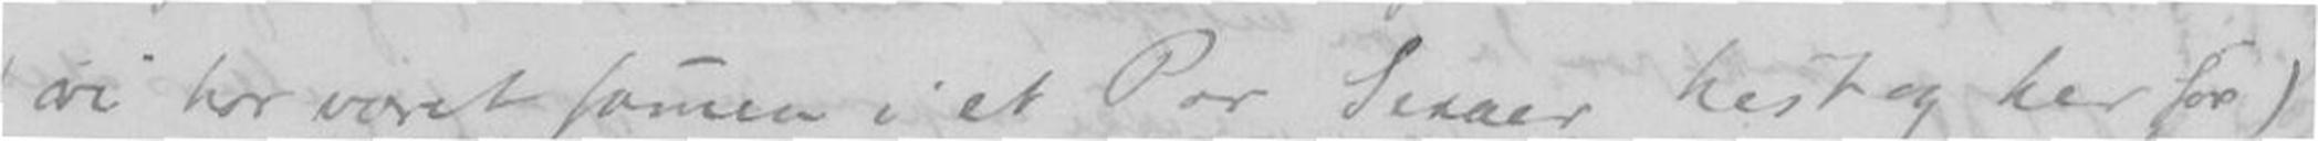(vi har været samen i et Par Steder hist og her før)
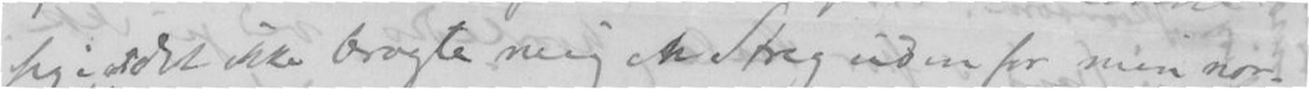sige at det ikke bragte mig et Steg udenfor min nor-
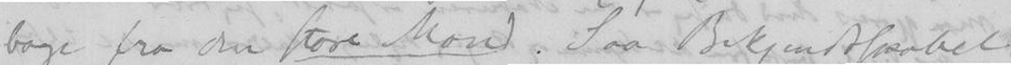bage fra den store Mand. Saa Bekjendtskabet
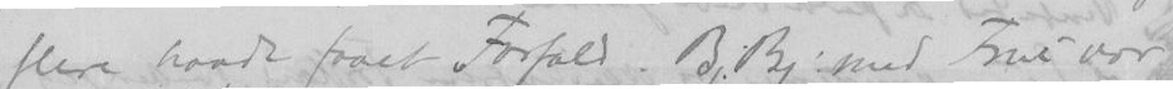flere havde faaet Forfald. Bj.Bj. med Frue var
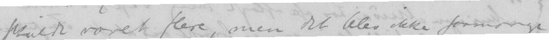skulde været flere, men det blev ikke saamange,
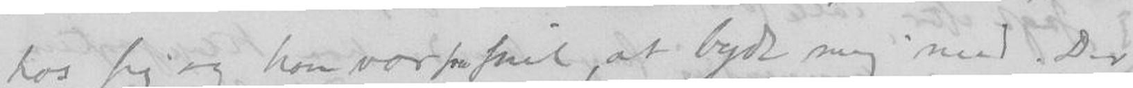hos sig og han var saa snild, at byde mig med. Der
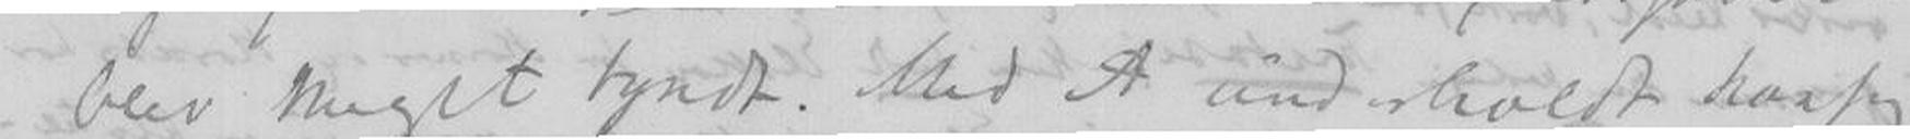blev Meget tyndt. Med A underhold han sig
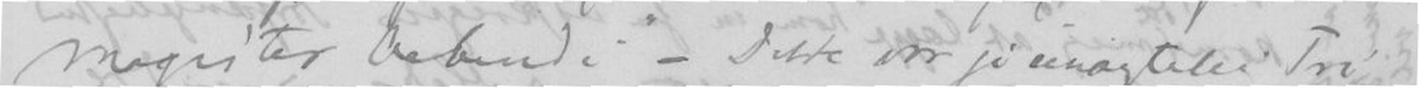Magister Aabent. - Dette var jo unægtelse Tri-
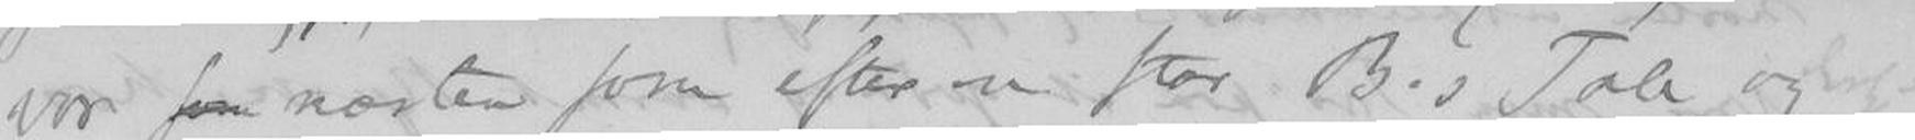var næsten som efter en stor B.s Tale og
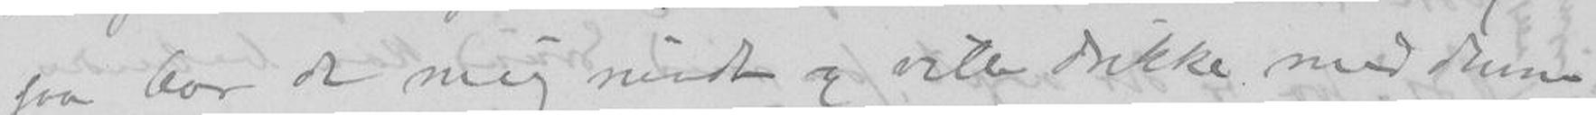saa var de mig nødt og ville drikke med denne
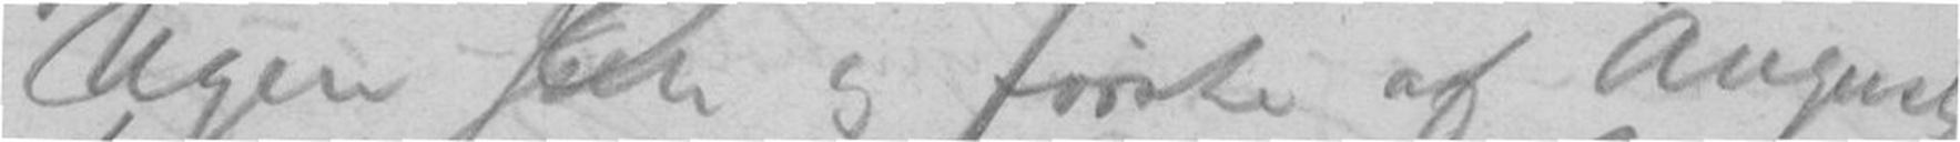Ugen Juli og første af August
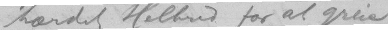hærdet Helbred for at greie
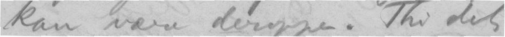kan være deroppe. Thi det
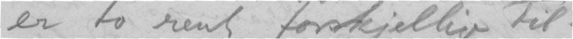er to rent forskjellige Til-
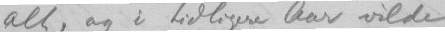alt, og i tidligere Aar vilde
